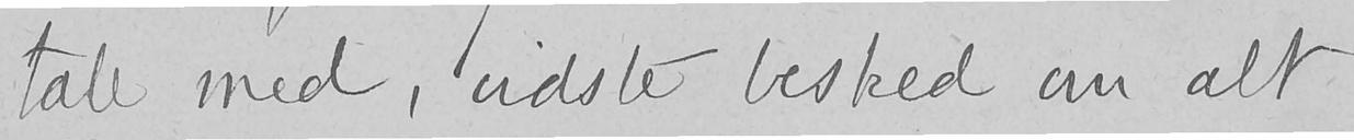tale med, vidste besked om alt
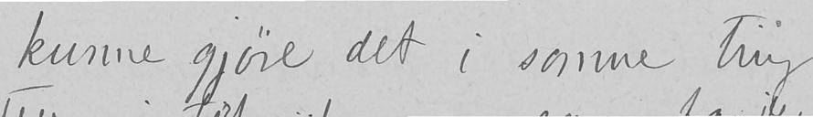kunne gjøre det i somme ting
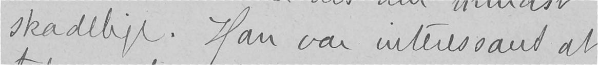skadelige. Han var interessant at
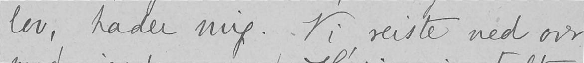lov, hader mig. Vi reiste ned over
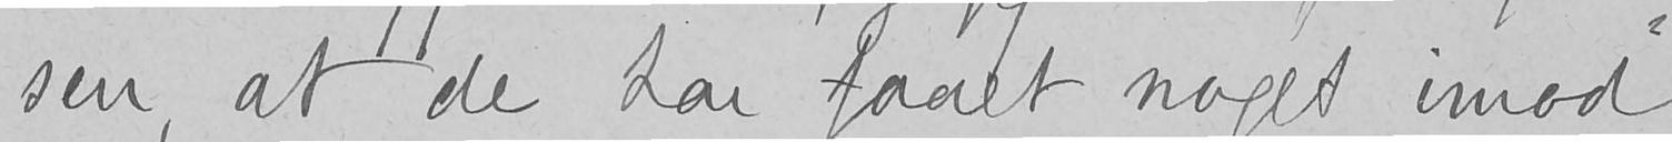sen, at de har faaet noget imod
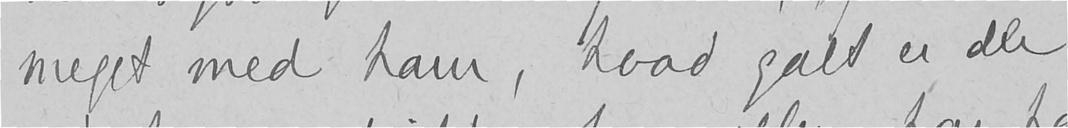meget med ham, hvad galt er der
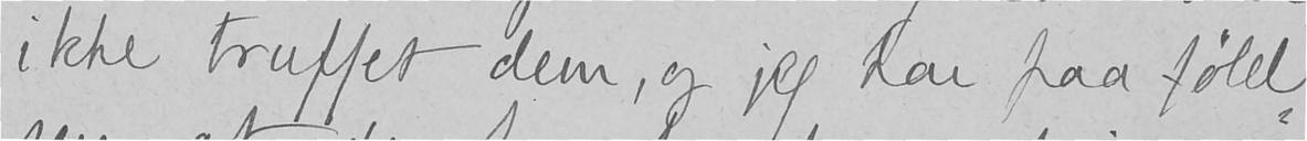ikke truffet dem, org jeg har paa følel-
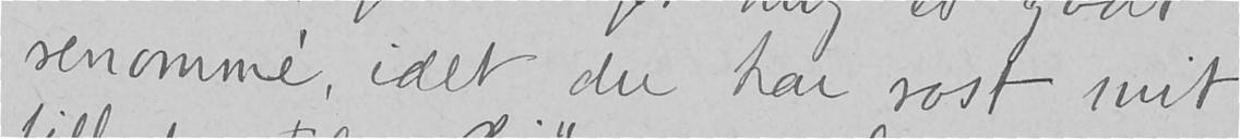renomme, idet du har rost mit
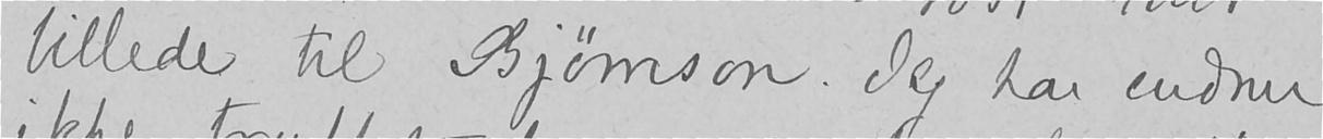billede til Bjørnson. Jeg har endnu
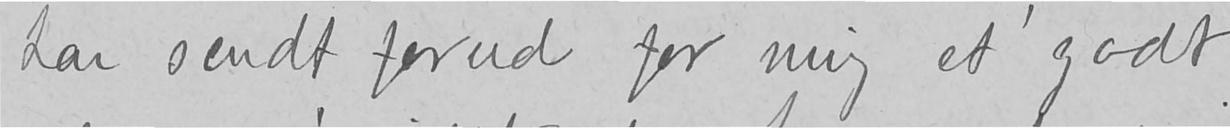har sendt forud for mig et godt
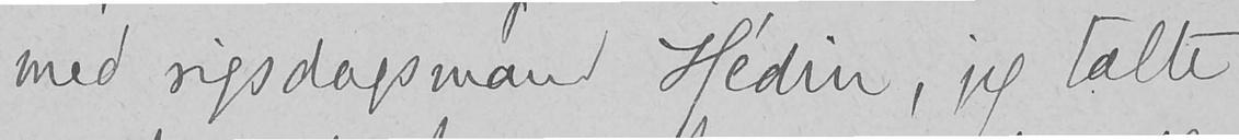med rigsdagsmand Hedin, jeg talte
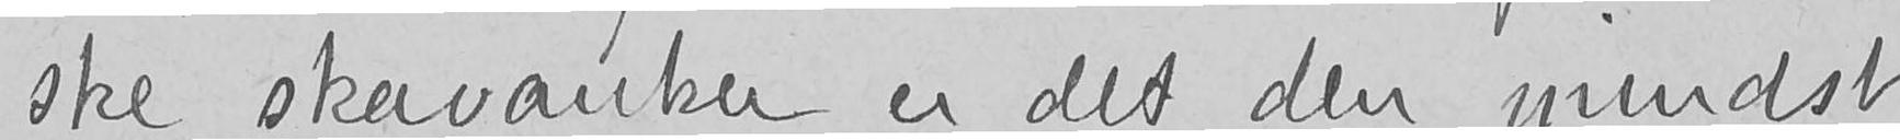ske skavanker er det den mindst
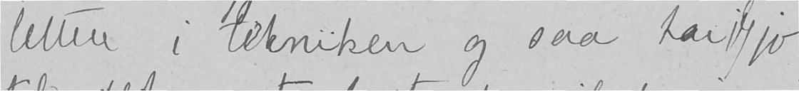lettere i tekniken og saa har jeg jo
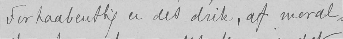Forhaabentlig er det drik, af moral-
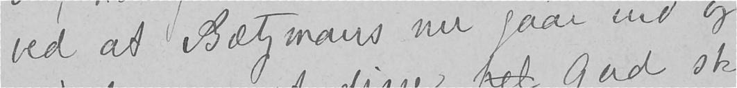ved at Bætzmans nu gaar ind og
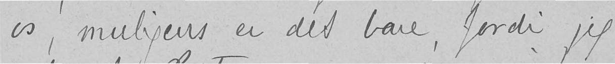os, muligens er det bare, fordi jeg
